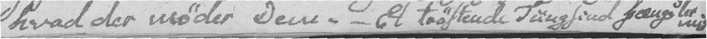hvad der møder Dem. – Et trøstende Tungsind fængsler mig.
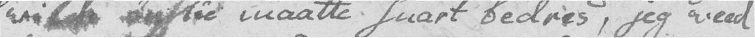vilde ønske maatte snart bedres, jeg veed
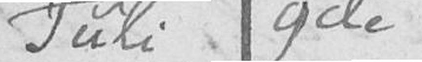Juli 9de
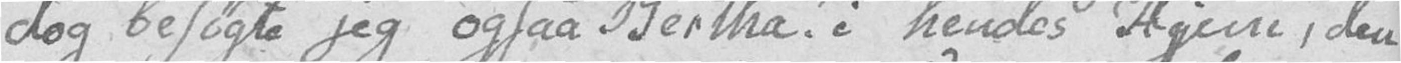dog besøgte jeg ogsaa Bertha! i hendes Hjem, den
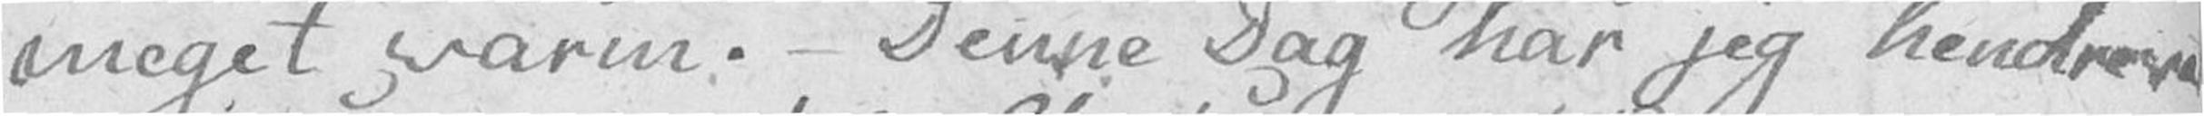meget varm. – Denne Dag har jeg hendreve
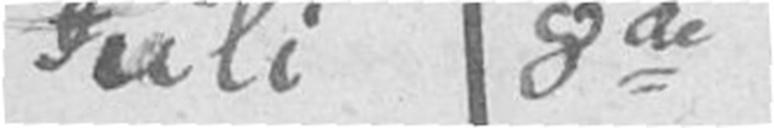Juli 8de
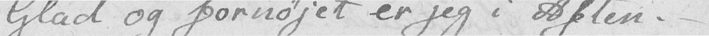Glad og fornøjet er jeg i Aften. –
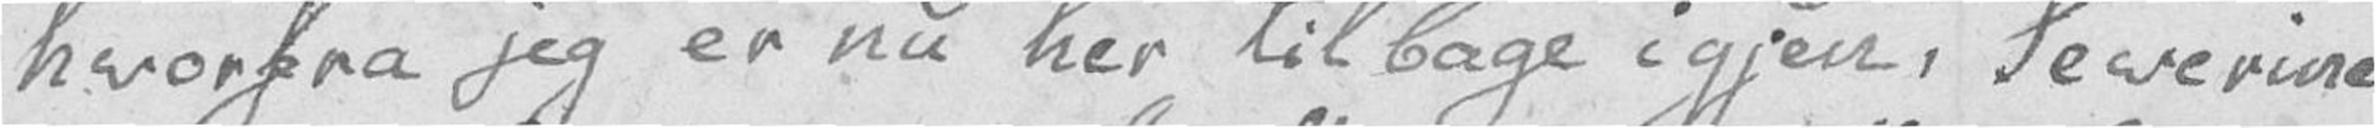hvorfra jeg er nu her tilbage igjen, Severine
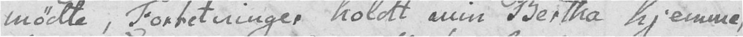mødte, Forretninger holdt min Bertha hjemme,
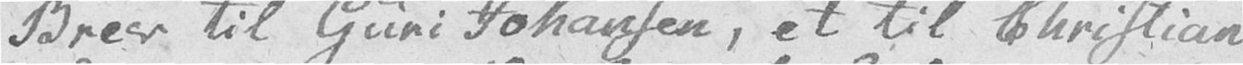Brev til Guri Johansen, et til Christian
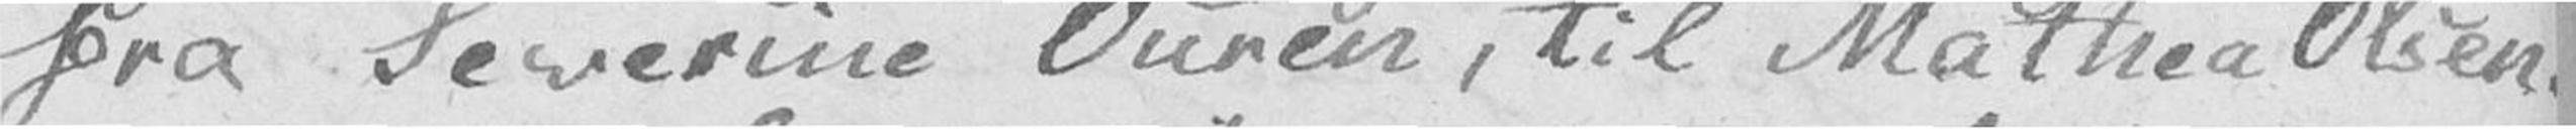fra Severine Ouren, til Mathea Olsen.
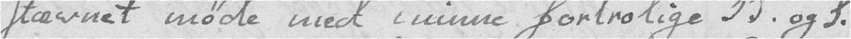stævnet møde med minne fortroelige B. og S.
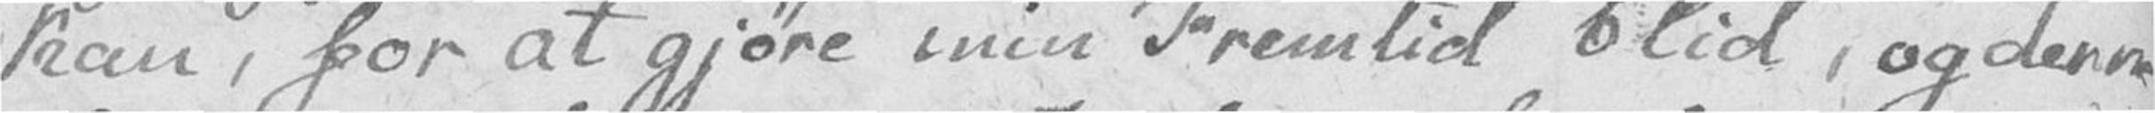kan, for at gjøre min Fremtid blid, og denne
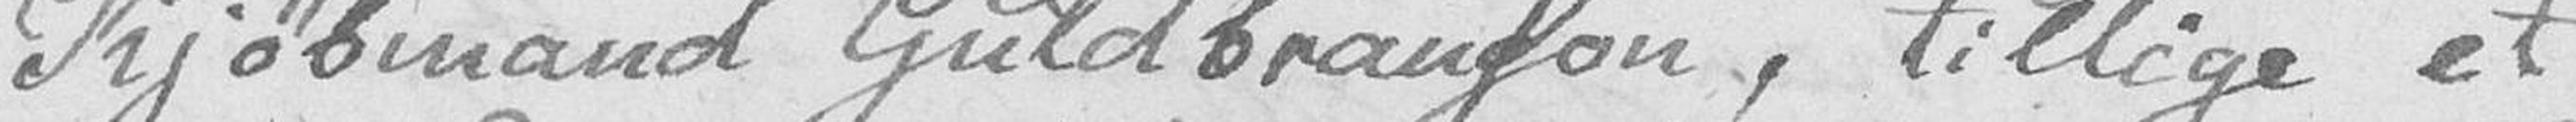Kjøbmand Guldbranson, tillige et
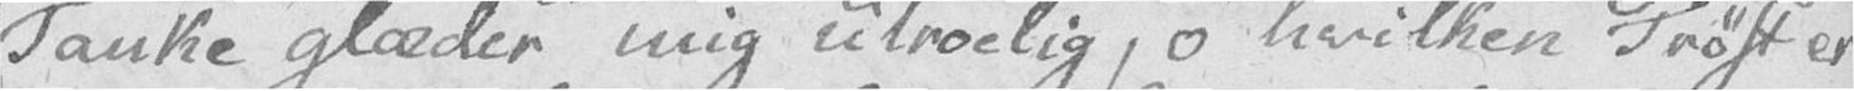Tanke glæder mig utroelig, o hvilken Trøst er
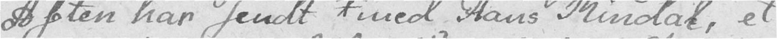Aften har sendt l med Hans Rindal, et
